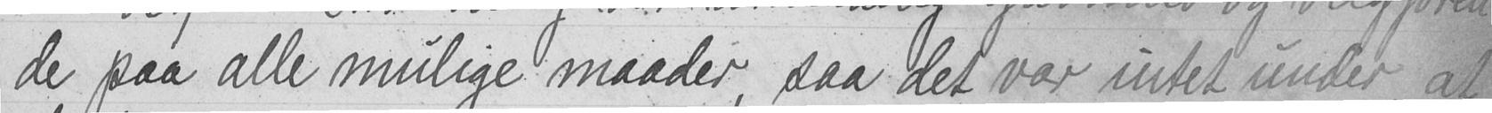de paa alle mulige maader, saa det var intet under, at
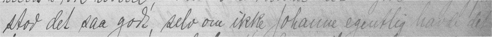stod det saa godt, selv om ikke Johanne egentlig havde det
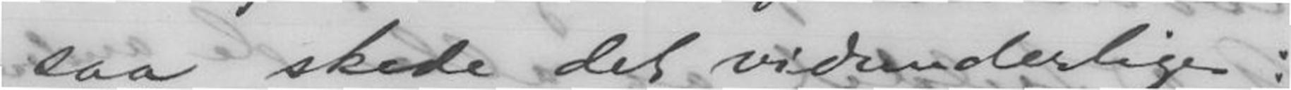saa skede det vidunderlige:
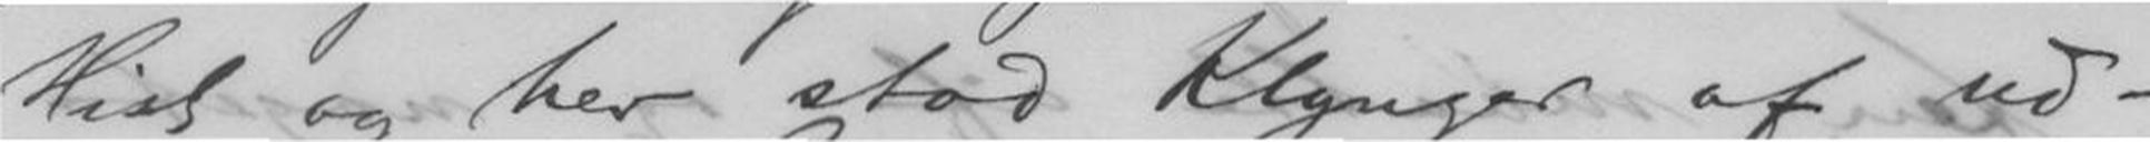Hist og her stod Klynger af ud-
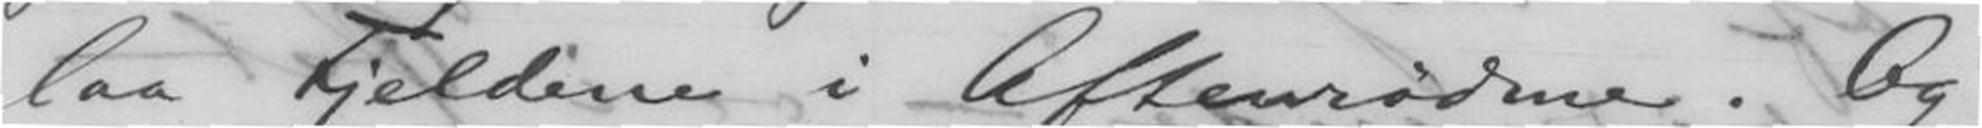laa Fjeldene i Aftenrødme. Og
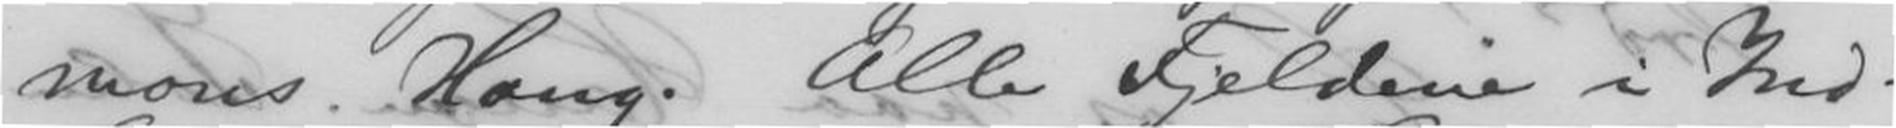mons Haug. Alle Fjeldene i Ind-
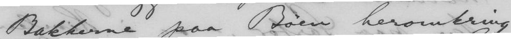Bakkerne paa Bøen heromkring
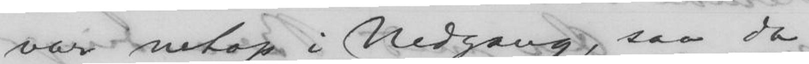var netop i Nedgang, saa da
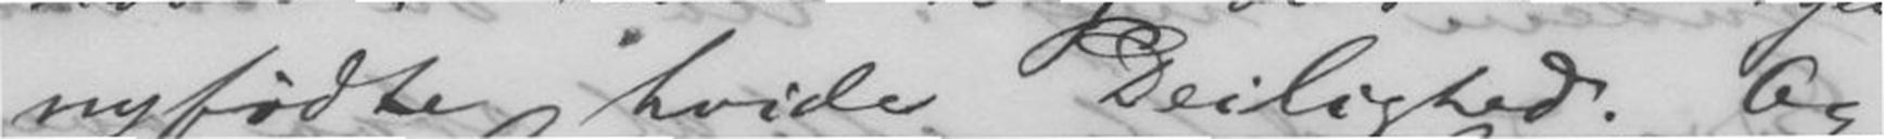nyfødte hvide Deilighed. Og
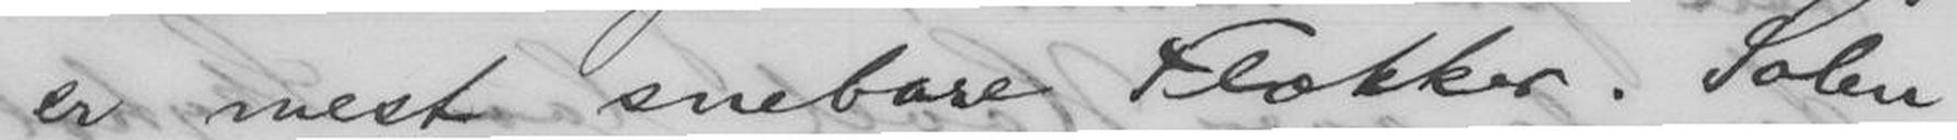er mest snebare Flækker. Solen
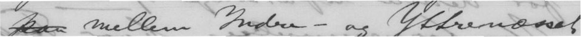paa mellem Indre- og Yttrenæsset
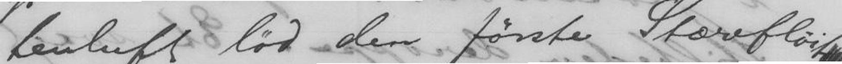tenluft lød den første Stærefløit !
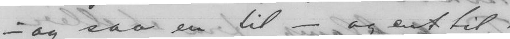- og saa en til - og en til -
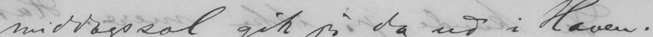middagsol gik jeg da ud i Haven.
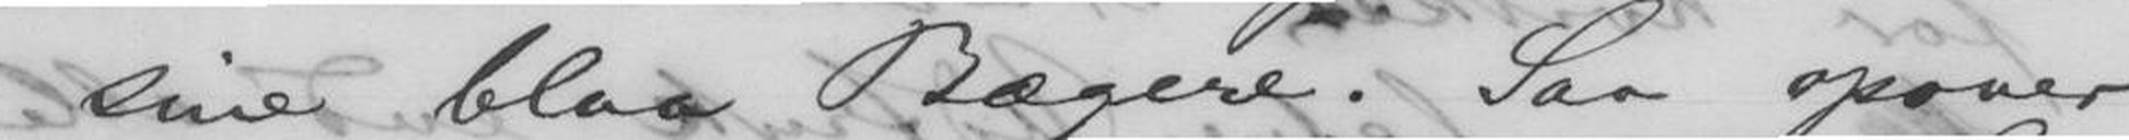sine blaa Bægere. Saa opover
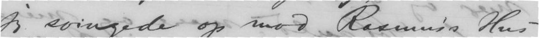jeg svingede op mod Rasmus's Hus
